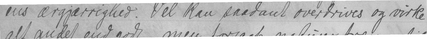ens ærgjærrighed. Vel kan saadant overdrives og virke
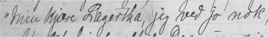"Min kjære Lagertha, jeg ved jo nok,
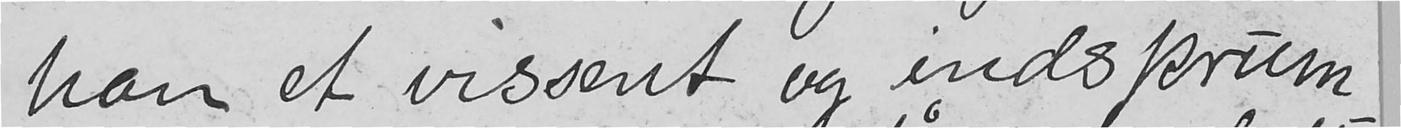han et vissent, og indskrum-
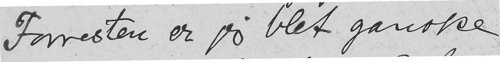Forresten er jeg blet ganske
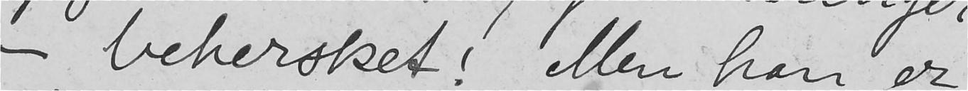- behersket! Men han er
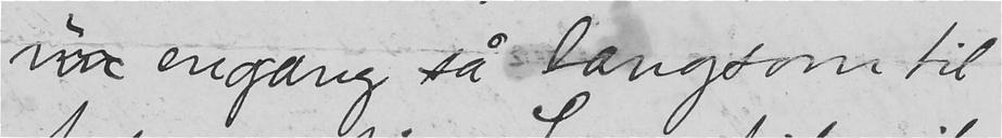nu engang så langsom til
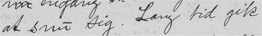at snu sig. Lang tid gik
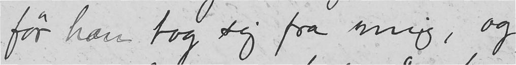før han tog sig fra mig, og
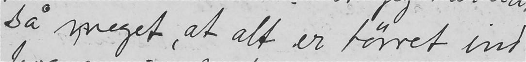så meget, at alt er tørret ind
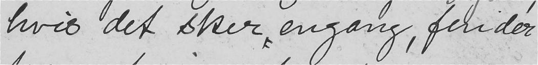hvis det sker engang, finder
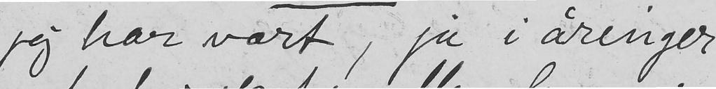jeg har vært, ja i åringer
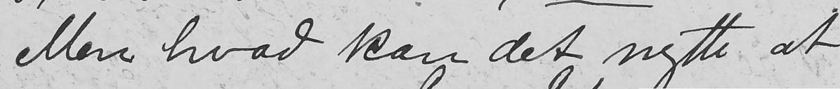Men hvad kan det nytte at
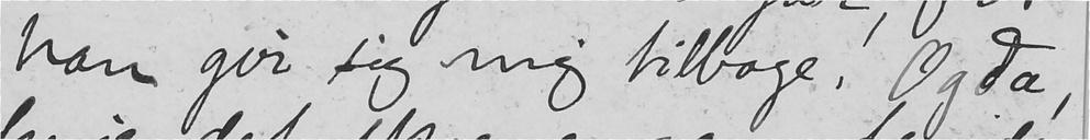han gir sig mig tilbage! Og da,
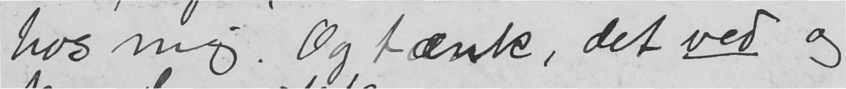hos mig. Og tænk, det ved og
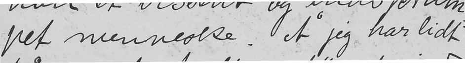pet menneske. Å jeg har lidt
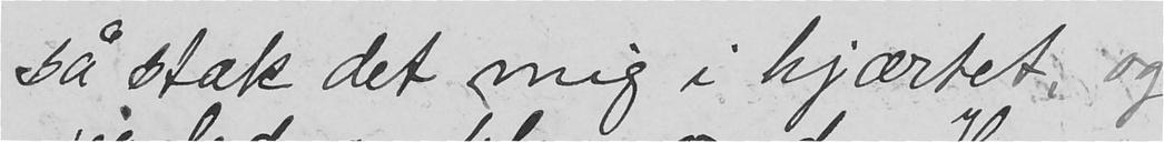så stak det mig i hjærtet, og
